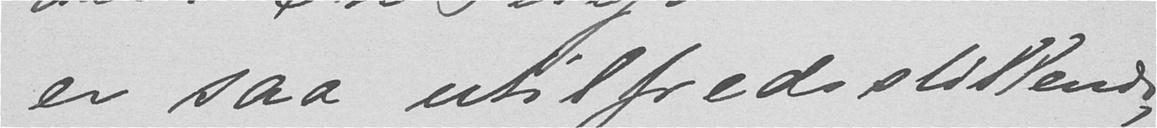er saa utiltredsstillende,
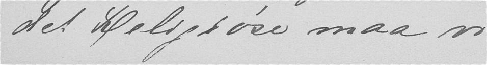det Religiøse maa vi
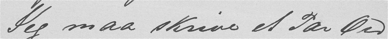Jeg maa skrive et Par Ord
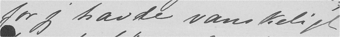for jeg havde vanskeligt
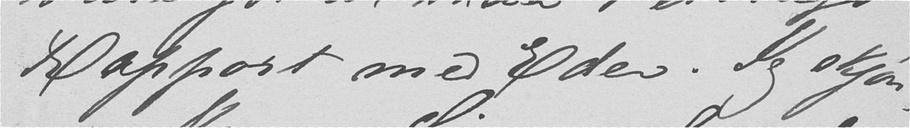Rapport med Eder. Jeg skjøn-
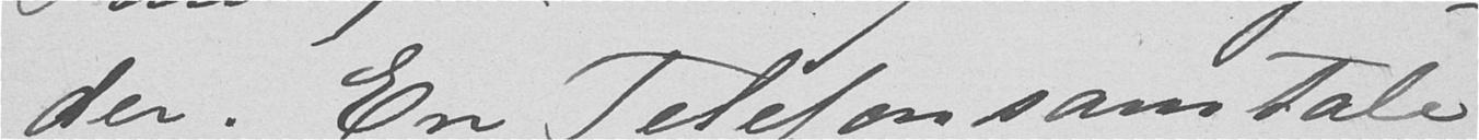der. En Telefonsamtale
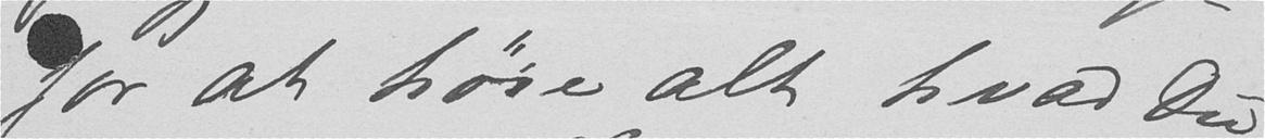for at høre alt hvad du
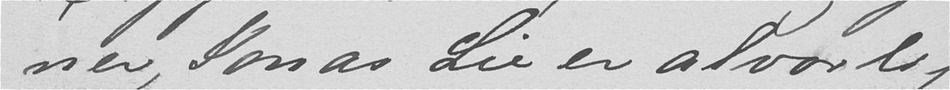ner, Jonas Lie er alvorlig
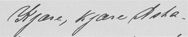Kjære, Kjære Asta.
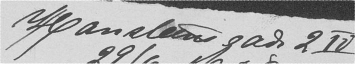Hansteens gade 2 IV
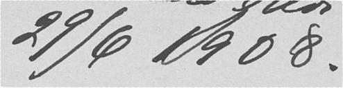29/6 1908.
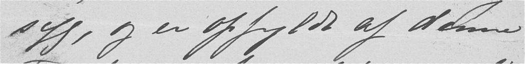syg, og er opfyldt af denne
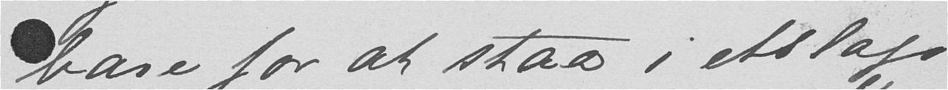bare for at staa i etslags
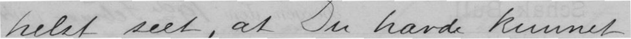helst seet, at Du havde kunnet
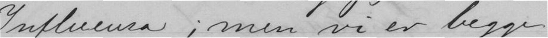Influenza; men vi er begge
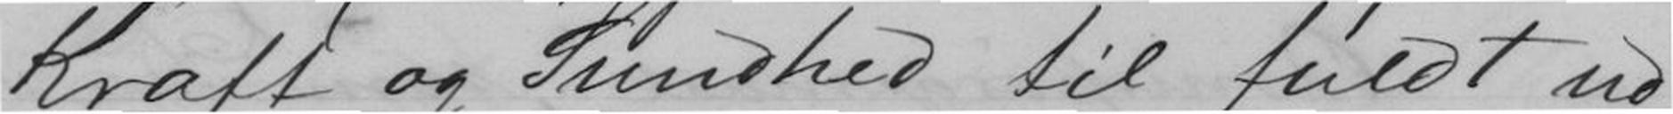Kraft og Sundhed til fuldt ud
